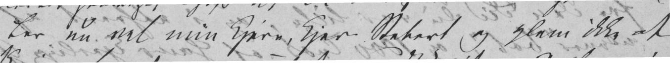Lev nu vel min kjere, kjere Robert og glem ikke at
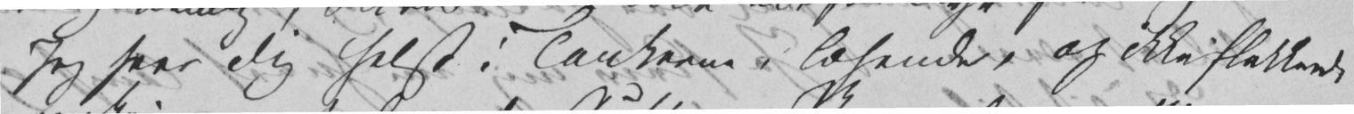Jeg seer Dig helst i Tankerne, læsende, og ikke flakkende
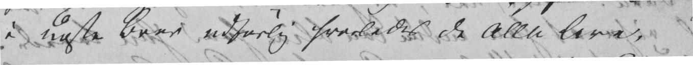i næste Brev udførlig hvorledes de Alle leve,
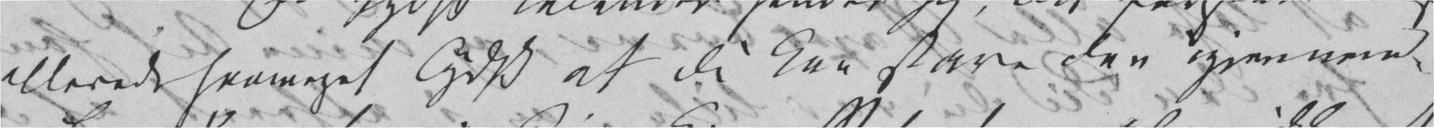allerede saameget Tydsk at Du kan stave den igjennem.
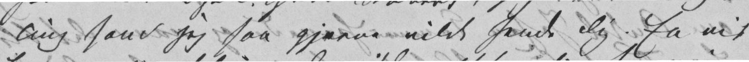Ting som jeg saa gjerne vilde sende Dig. En vis
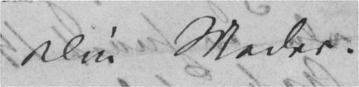Din Moder.
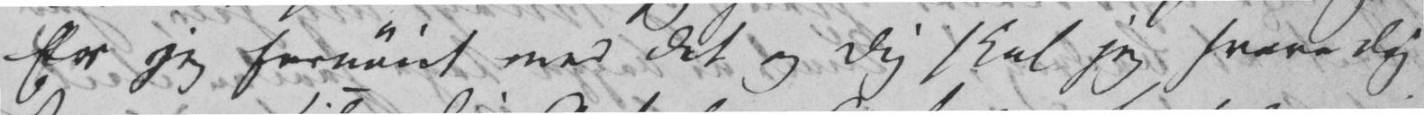Er jeg fornøiet med det og Dig skal jeg svare Dig
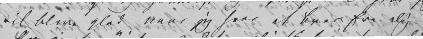vil blive glad naar jeg seer et Brev fra Dig.
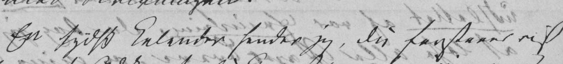En tydsk Kalender sender jeg, Du forstaaer vist
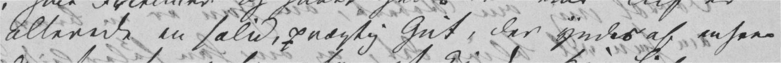allerede en solid, prægtig Gut, der yndes af enhver
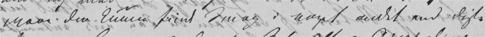maae Du kunne finde Smag i noget andet end disse
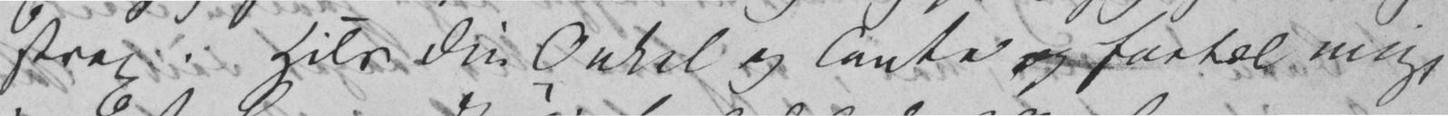strax. Hils Din Onkel og Tante og fortæl mig
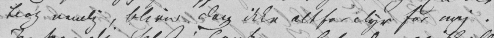Bog nemlig, bliver den ikke altfor dyr for mig.
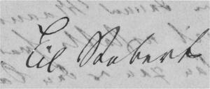Til Robert
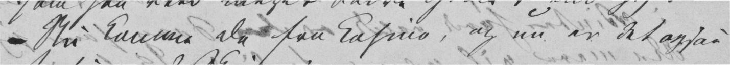– Nu komme de fra Kasino, og nu er det ogsaa
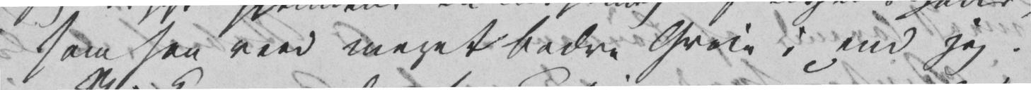som han veed meget bedre Greie i end jeg.
